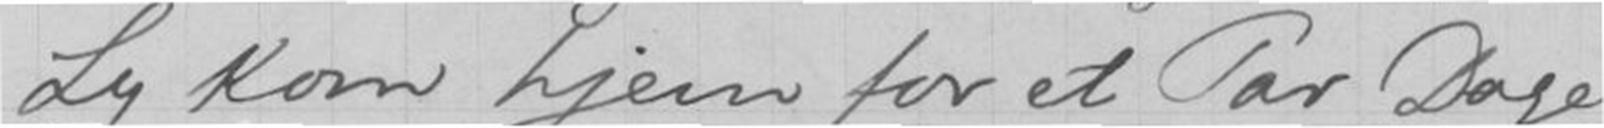Ly kom hjem for et Par Dage
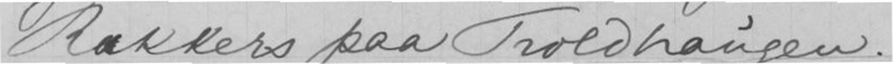Rakkers paa Troldhaugen.
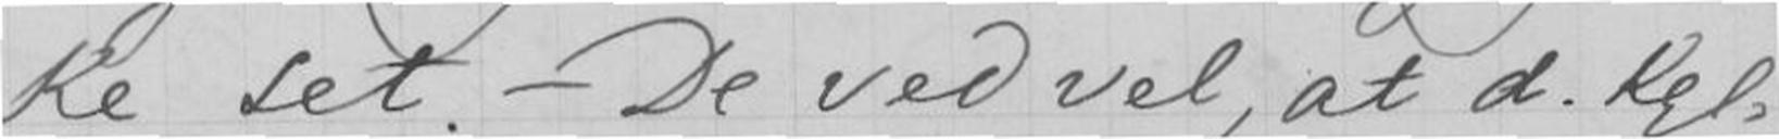ke set. - De ved vel, at d. Kgl.
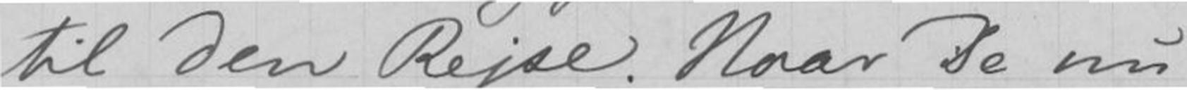til den Rejse. Naar De nu
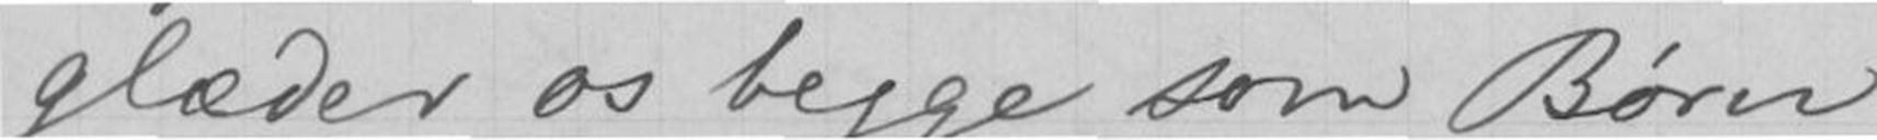glæder os begge som Børn
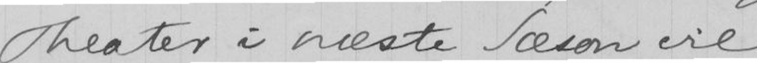Theater i neste Sæson vil
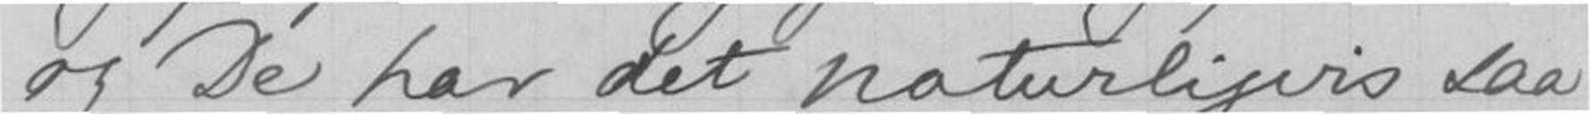og De har det naturligvis saa
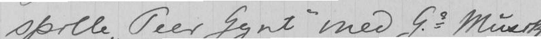spille Peer Gynt med G.s Musik.
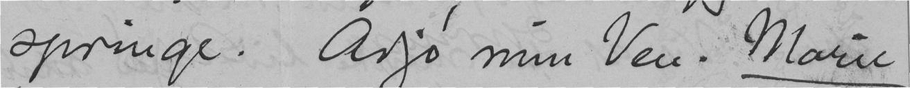springe. Adjø min Ven. Marie
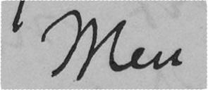Men
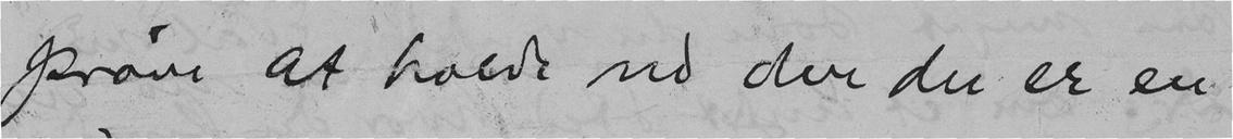prøve at holde ud der du er en
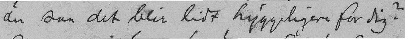du saa det blir lidt hyggeligere for dig?
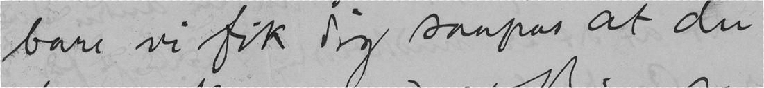bare vi fik dig saapas at du
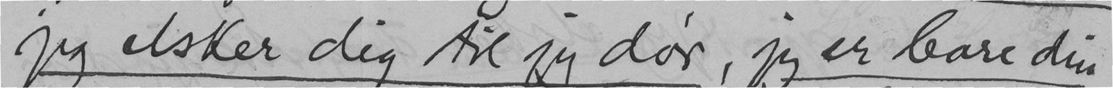jeg elsker dig til jeg dør, jeg er bare din
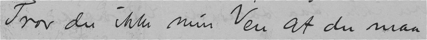Tror du ikke min Ven at du maa
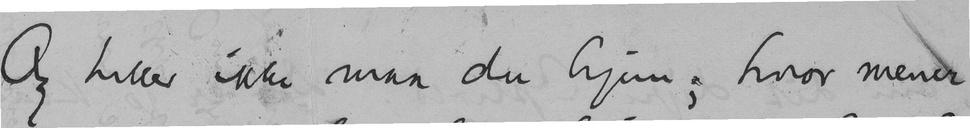Og heller ikke maa du hjem; hvor mener
